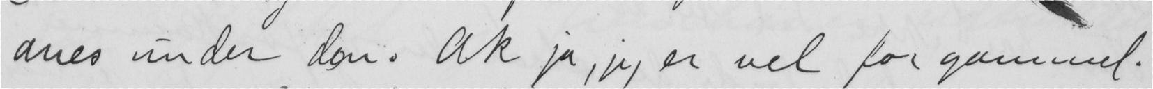anes under den. Ak ja, jeg er vel for gammel.
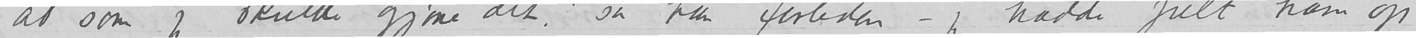ad som jeg skulde gjøre det.» sa han forleden – jeg hadde jult ham op
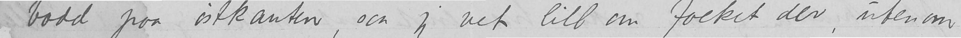bodd paa østkanten, saa jeg vet litt om folket der, utenom
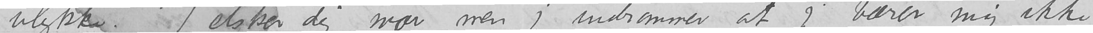ulykke.  «Jeg elsker dig, mor, men jeg indrømmer at jeg bærer mig ikke
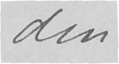din
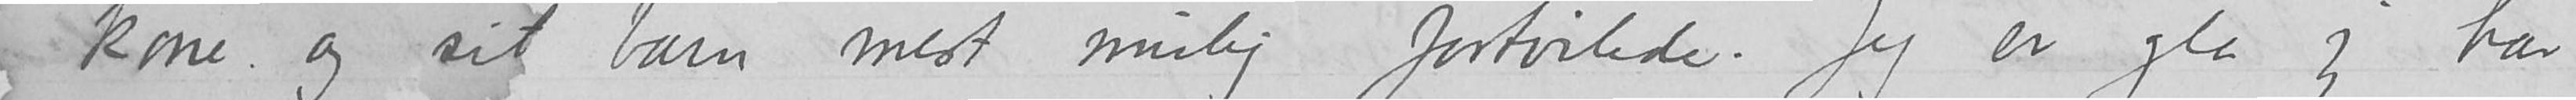kone og sit barn mest mulig fortvilede. Jeg er gla jeg har
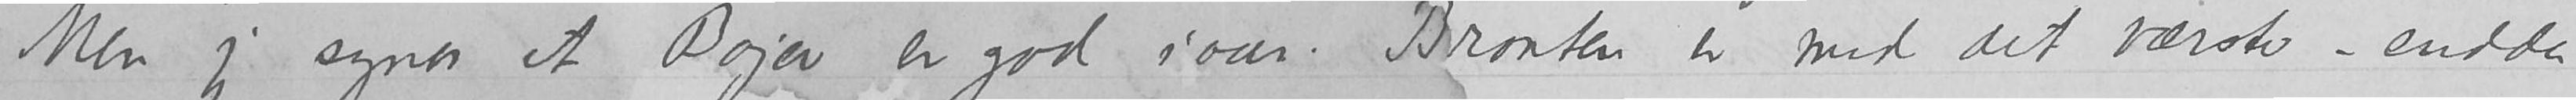Men jeg synes at Bojer er god i aar.  Braaten er med det værste – endda
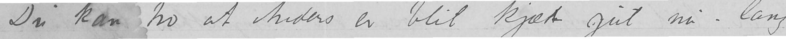Du kan tro at Anders er blitt kjæk gut nu – lang
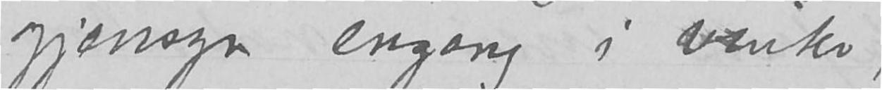gjensyn engang i vinter,
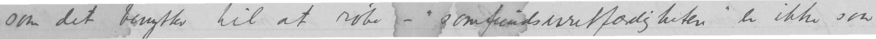som det benytter til at røbe –- «samfundsuretfærdigheten» er ikke saa
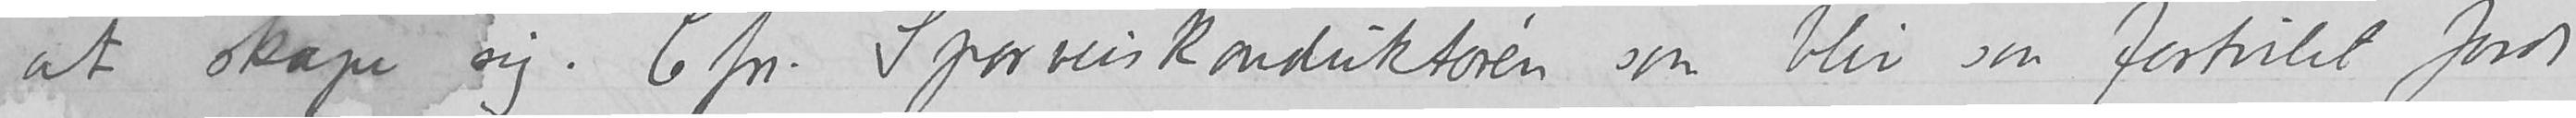at skape sig.Cfr Sporveiskonduktøren som blir saa fortvilet fordi
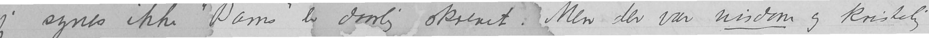jeg synes ikke «Bams» er daarlig skrevet. Men der var visdom og kristelig
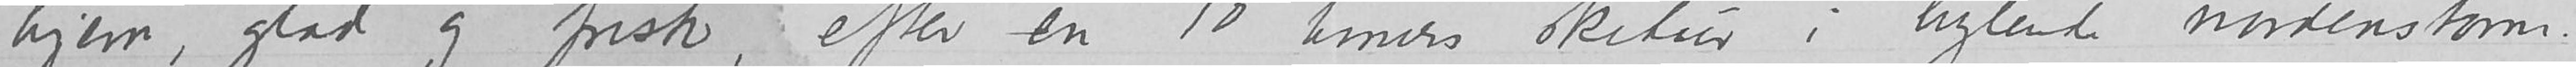hjem, glad og frisk, efter en 10 timers skitur i hylende nordenstorm.
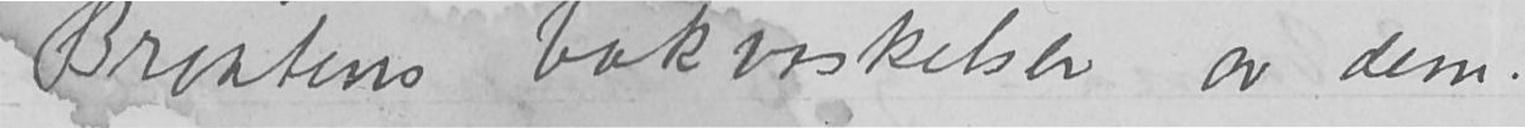Braatens bakvaskelser av dem.
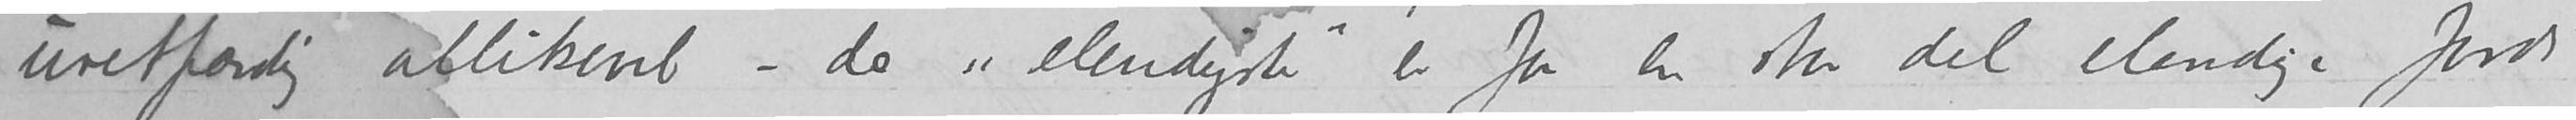uretfærdig allikevel – de «elendigste» er for en stor del elendige fordi
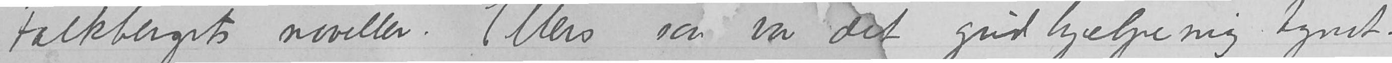Falkbergets noveller. Ellers saa var det gud hjelpe mig tyndnt
